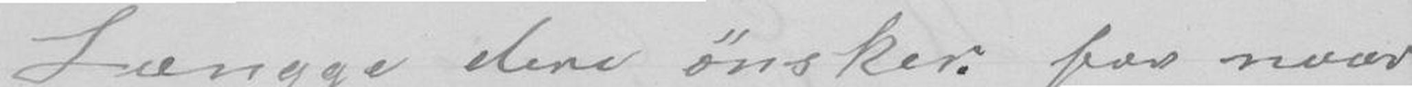Længge dere ønsker for naar
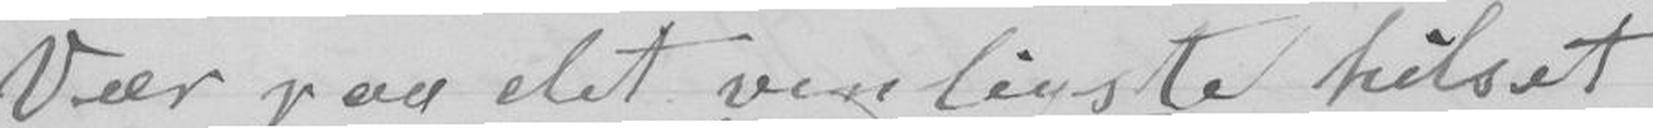Vær paa det venligste hilset
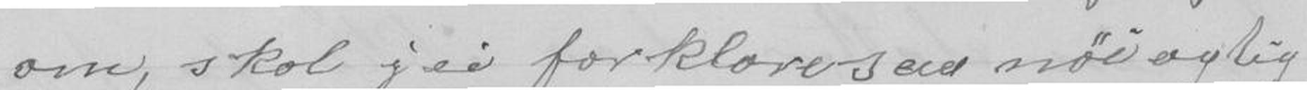om, skal jei forklare saa nøiagtig
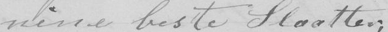mine beste Slaatter,
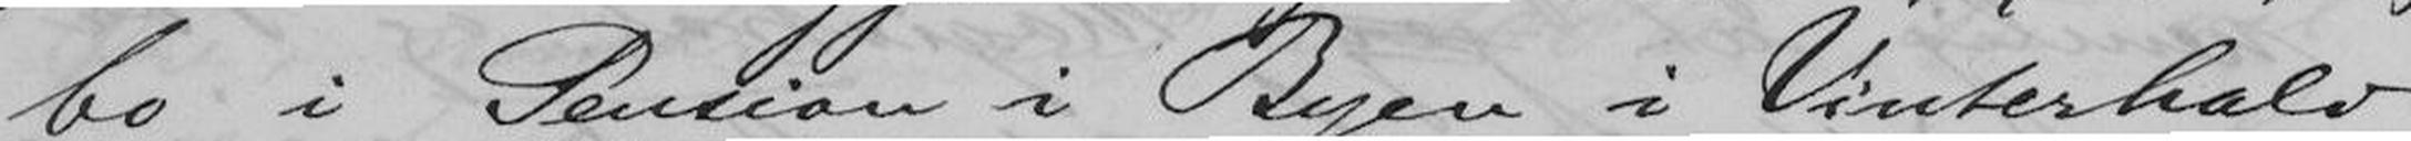bo i Pension i Byen i Vinterhalv-
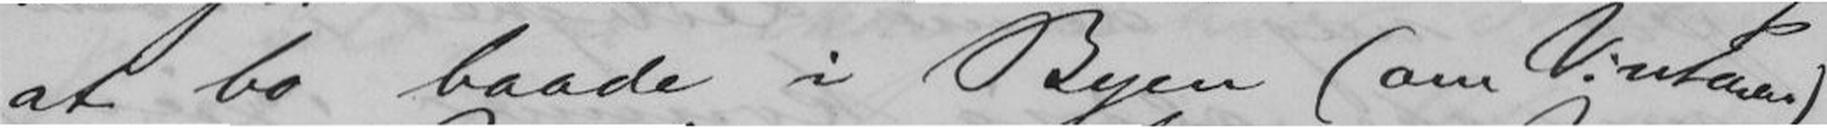at bo baade i Byen (om Vinteren)
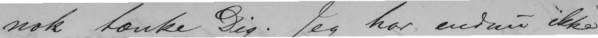nok tænke Dig. Jeg har endnu ikke
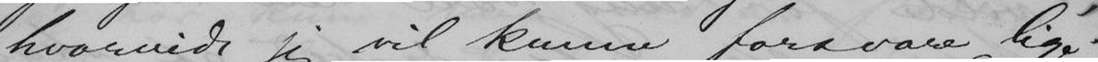hvorvidt jeg kunne forsvare lige
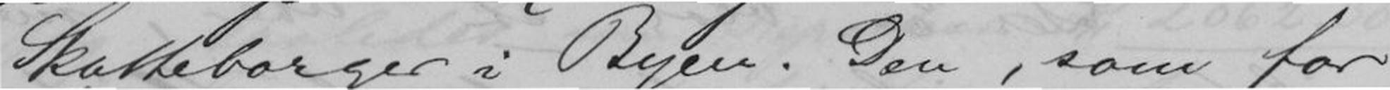Skatteborger i Byen. Den, som for
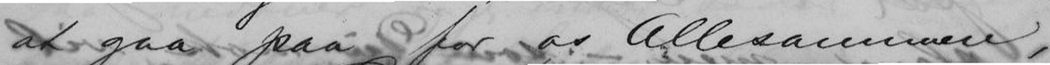at gaa paa for os Allesammen,
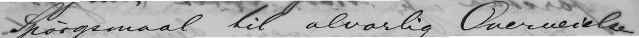Spørgsmaal til alvorlig Overveielse,
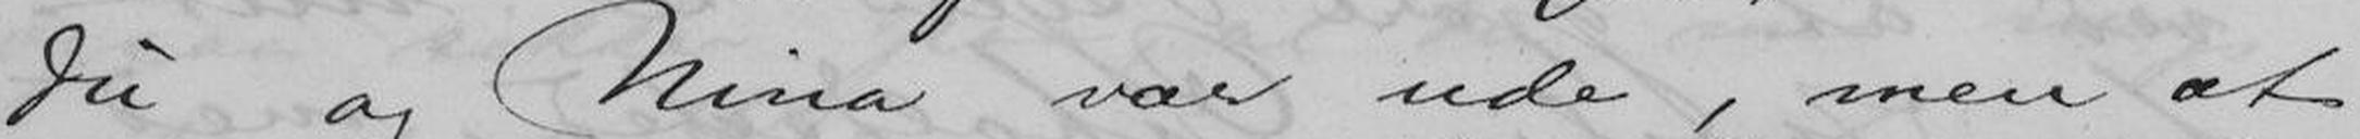Du og Nina var ude, men at
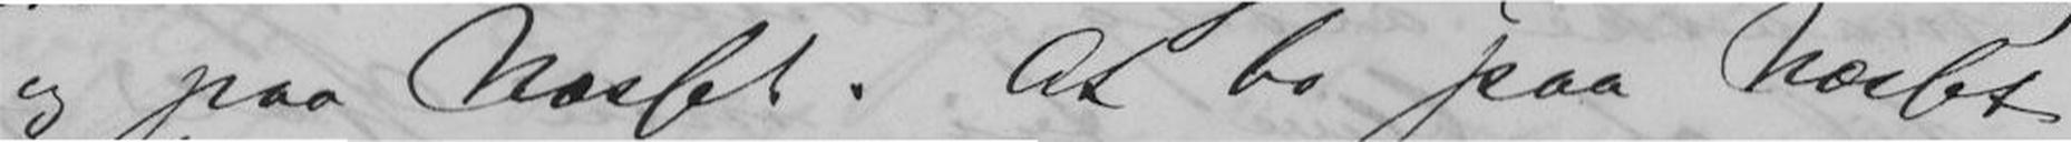og paa Næsset. At bo paa Næsset
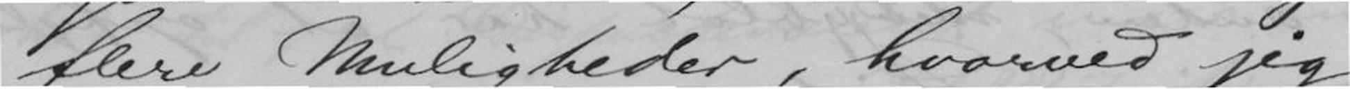flere Muligheder, hvorved jeg
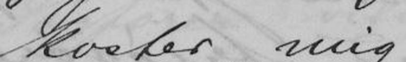koster mig:
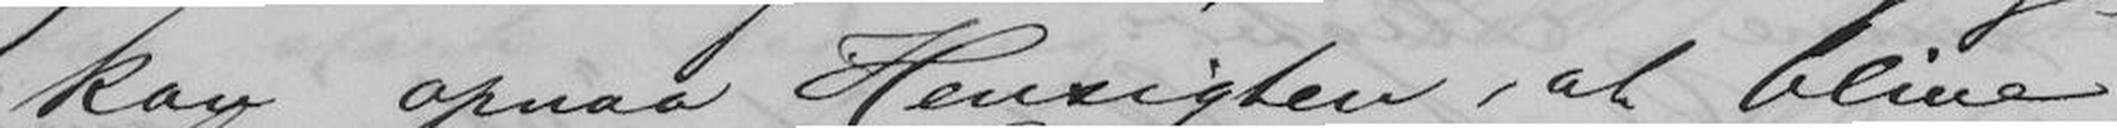kan opnaa Hensigten, at blive
